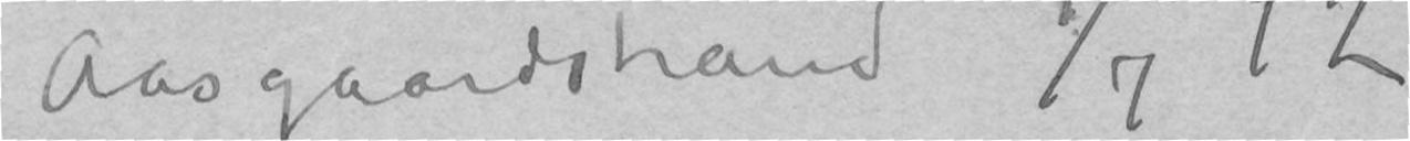Aasgaardstrand 9/7 92
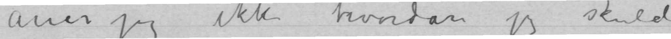aner jeg ikke hvordan jeg skulde
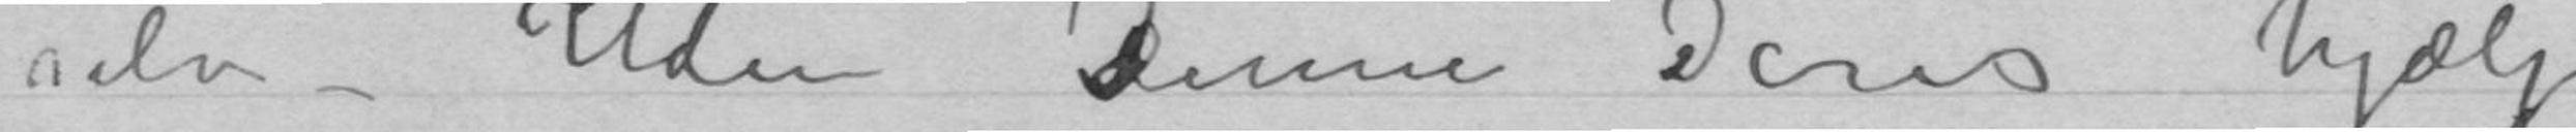selv – Uden denne Deres hjælp
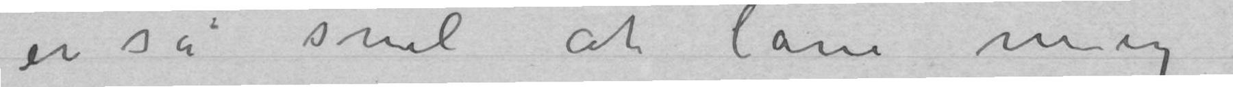er så snil at låne mig –
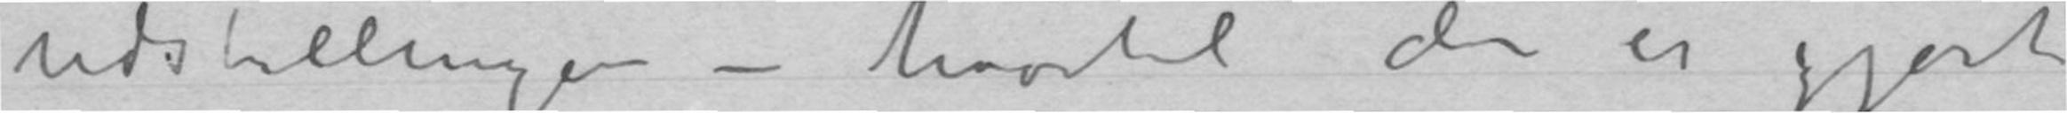udstillingen – hvortil der er gjort
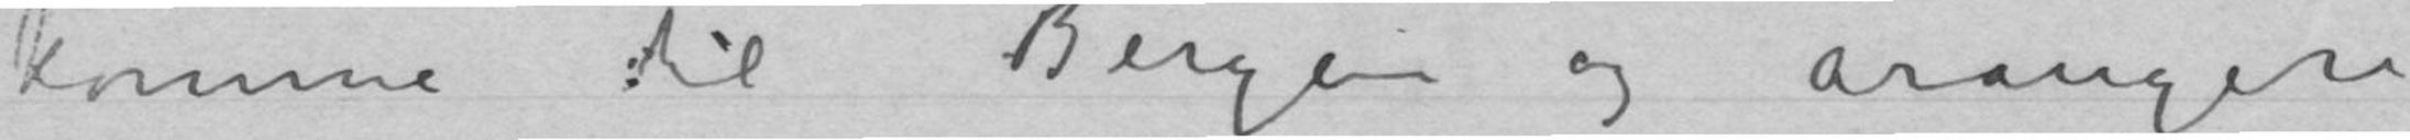komme til Bergen og arangere
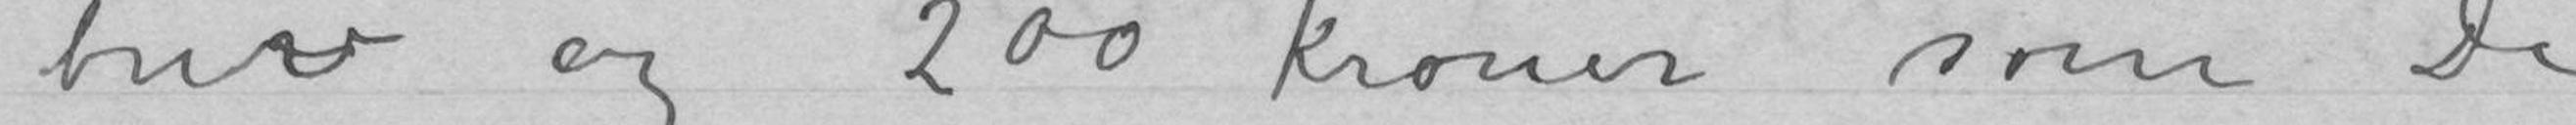brev og 200 kroner som De
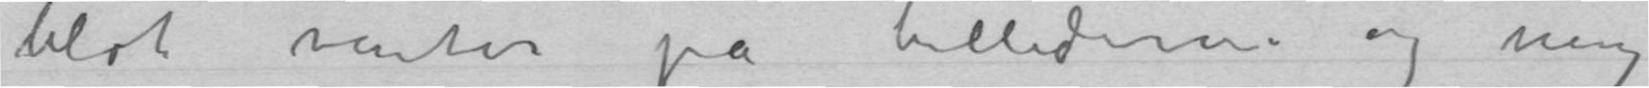blot venter på billederne og mig
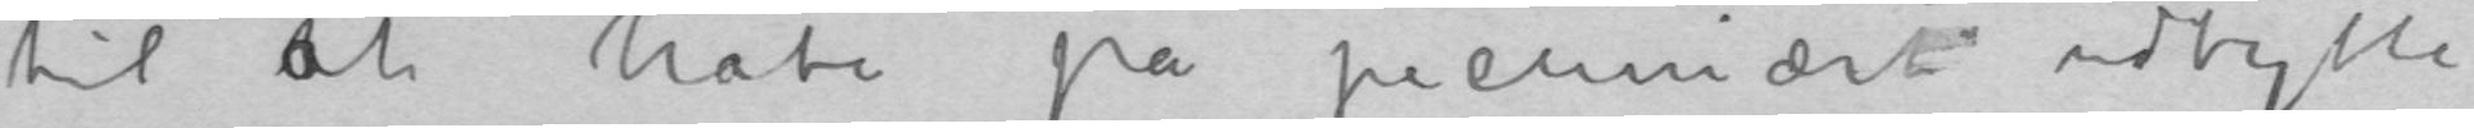til at håpe på pecuniært udbytte
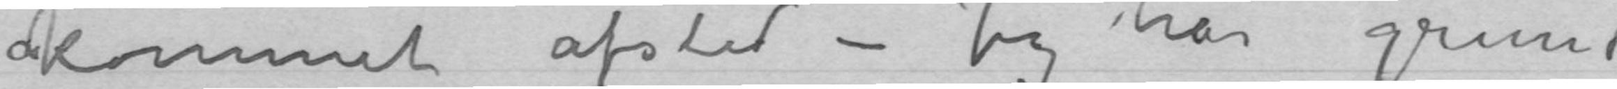kommet afsted – Jeg har grund
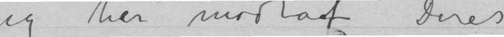Jeg har modtat Deres
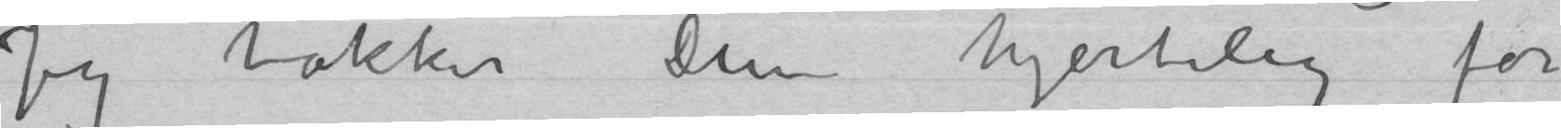Jeg takker Dem hjertelig for
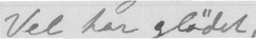Vel har glødet,
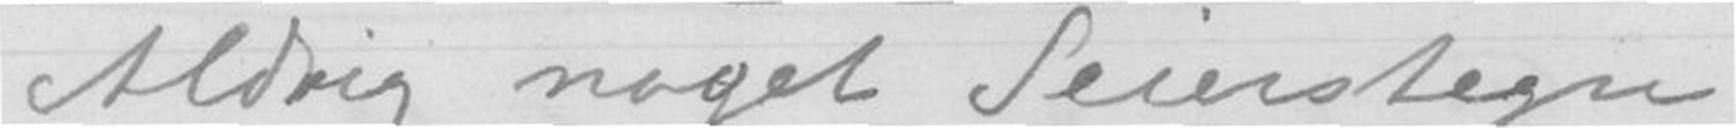Aldrig noget Seierstegn
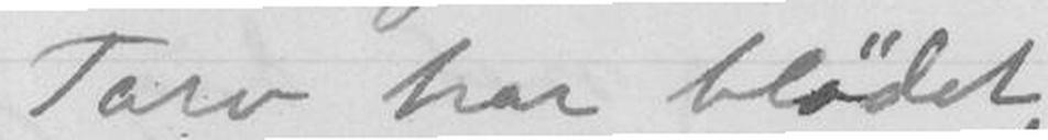Tarv har blødet,
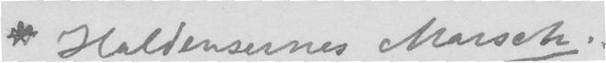* Haldensernes Marsch.
